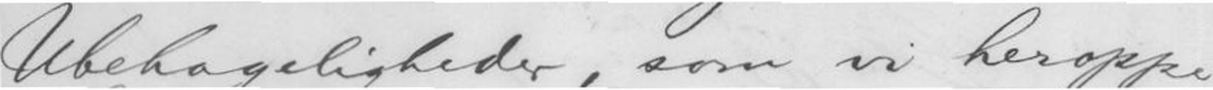Ubehageligheder, som vi heroppe
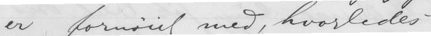er fornøiet med, hvorledes
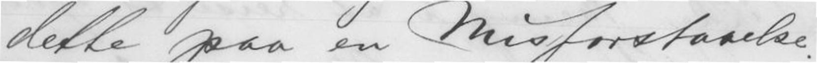dette paa en Misforstaaelse.
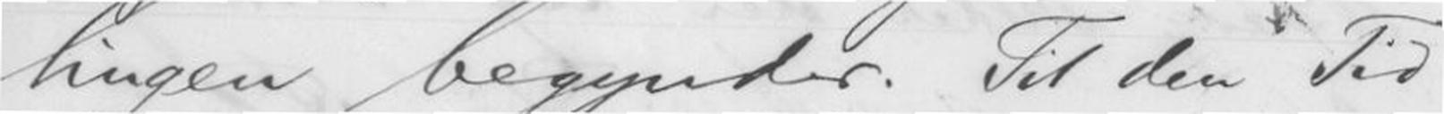lingen begynder. Til den Tid
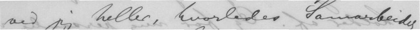ved jeg heller, hvorledes Samarbeidet
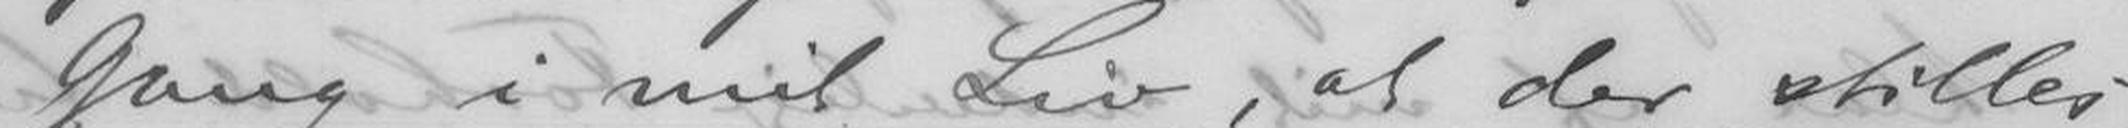Gang i mit Liv, at der stilles
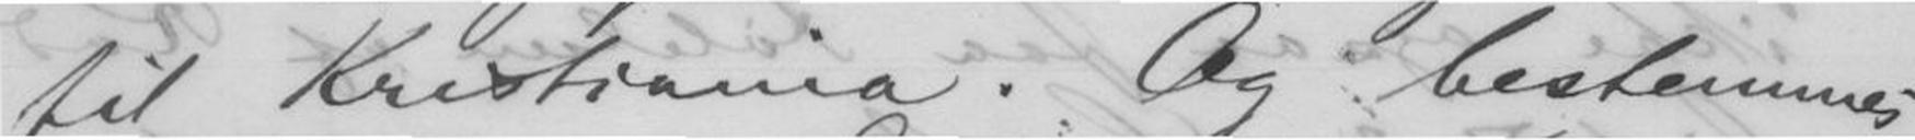til Kristiania. Og bestemmes
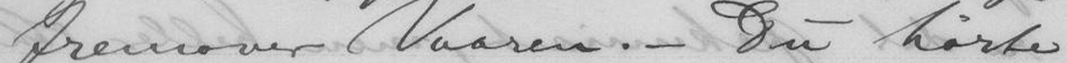fremover Vaaren. - Du hørte
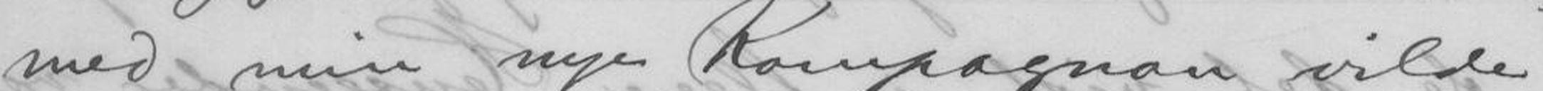med min nye Kompagnon vilde
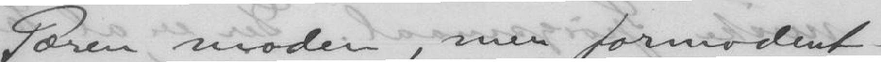Pæren moden, men formodent-
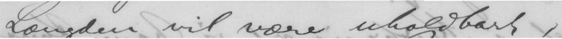Længden vil være uholdbart,
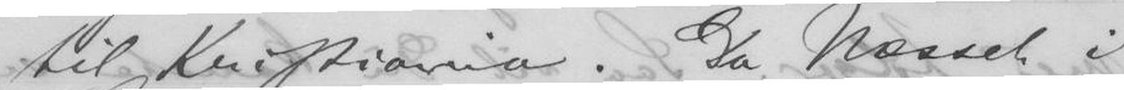til Kristiania. Da Næsset i
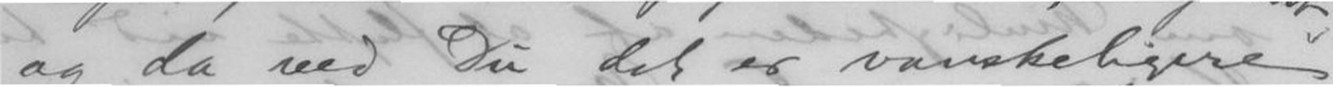og da ved Du det er vanskeligere
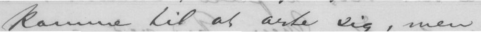komme til at arte sig, men
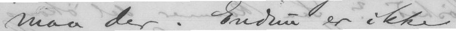maa der. Endnu er ikke
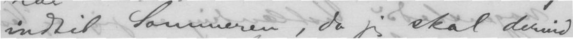indtil Sommeren, da jeg skal derind,
